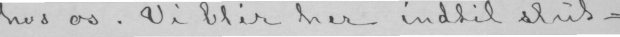hos os. Vi blir her indtil slut-
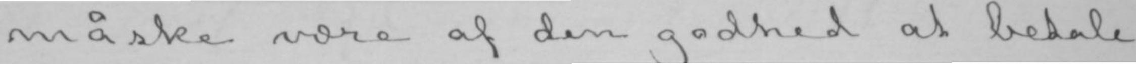måske være af den godhed at betale
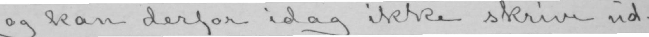og kan derfor idag ikke skrive ud-
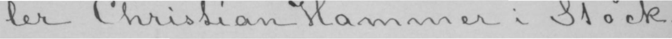ler Christian Hammer i Stock-
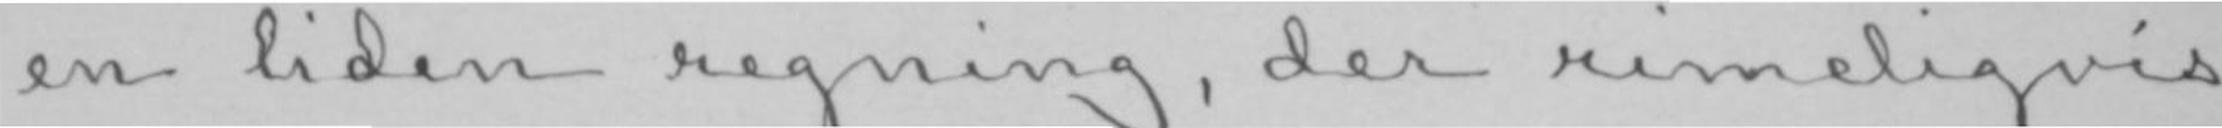en liden regning, der rimeligvis
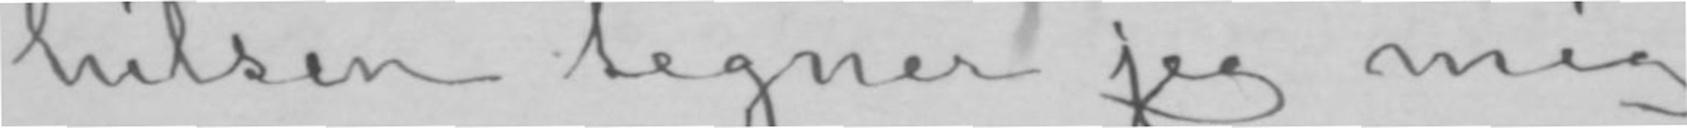hilsen tegner jeg mig
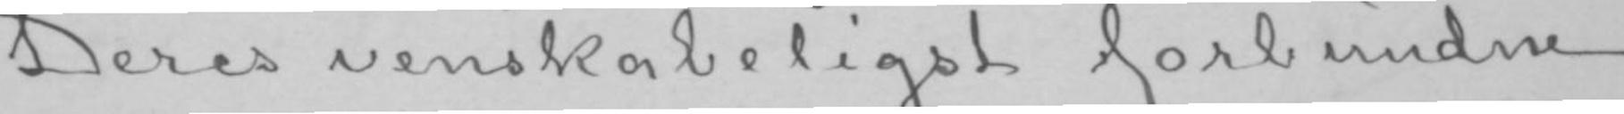Deres venskabeligst forbundne
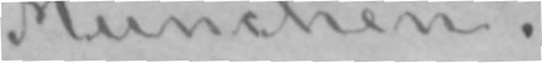München.
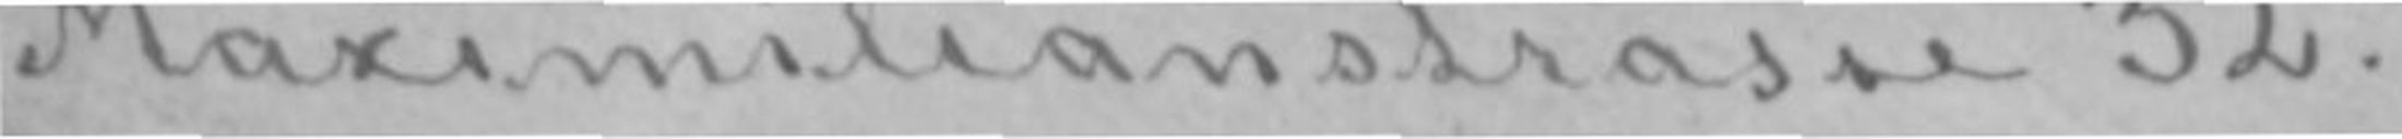Maximilianstrasse 32.
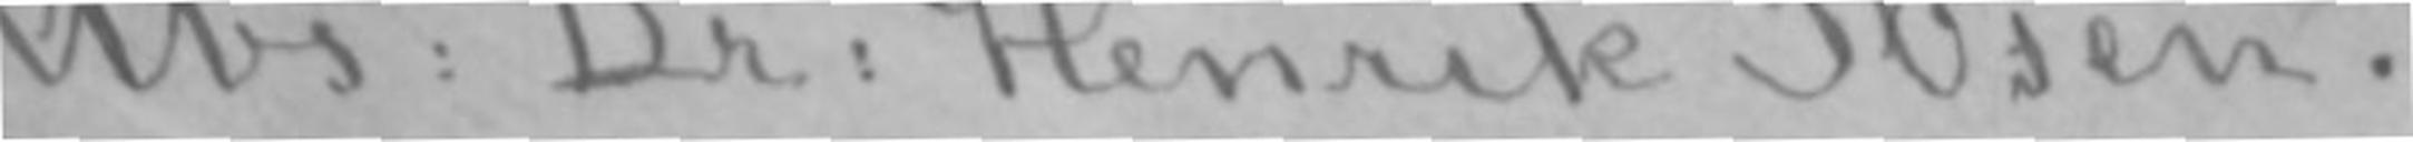Abs: Dr: Henrik Ibsen.
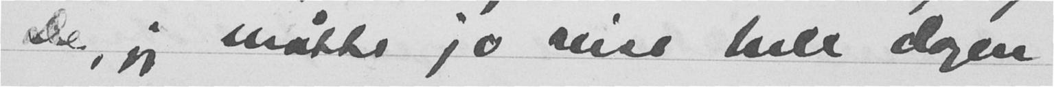De, jeg møtte jo reise hele dagen og
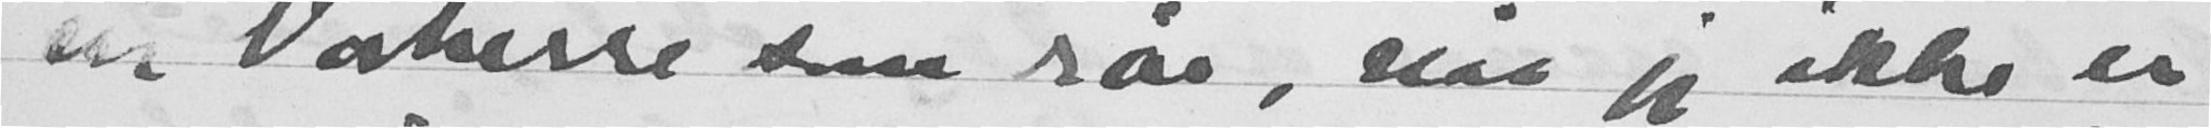er Vårherre som sår, når jeg ikke er
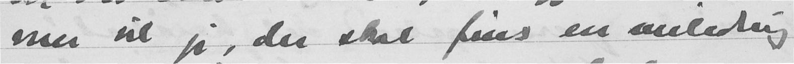mer vil jeg, der skal fins en veiledning
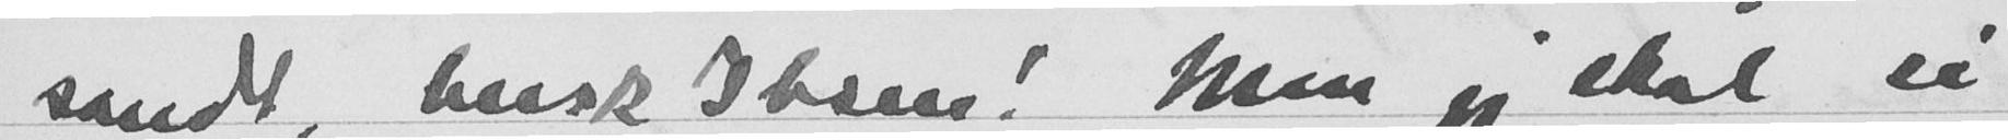sandt, husk Jesus! Men jeg skal si
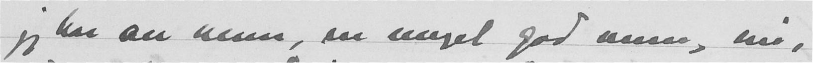jeg har en venn, en meget god venn, sier,
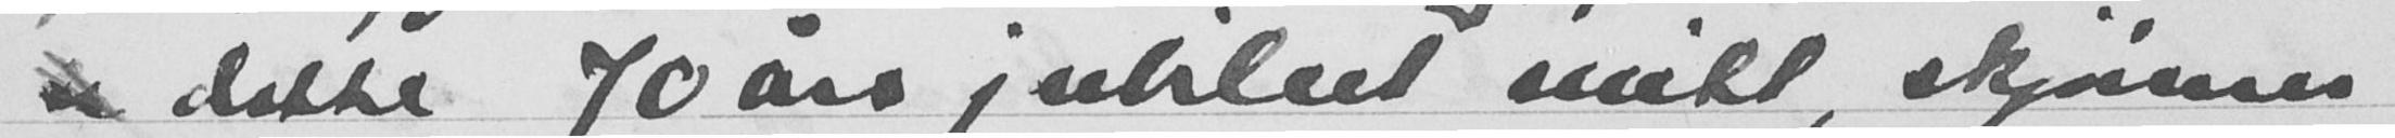dette 70 års jubileet mitt, skjønner
